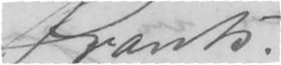Frants.
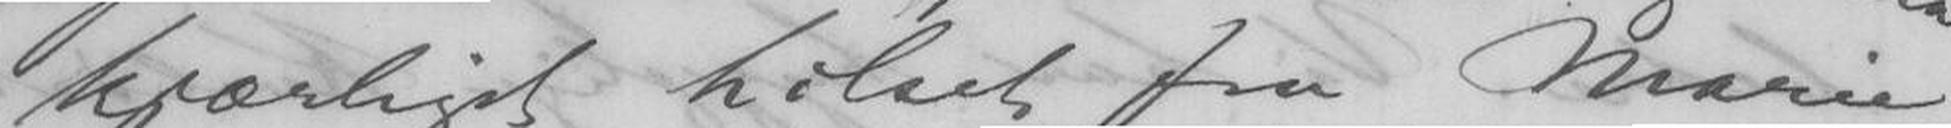kjærligst hilset fra Marie
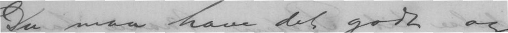Du maa have det godt og
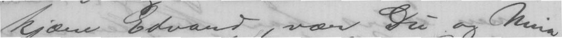kjære Edvard, vær Du og Nina
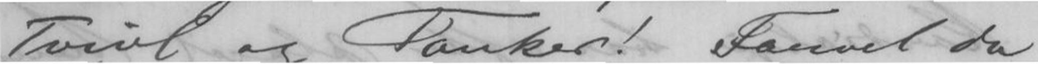Tvivl og Tanker! Farvel da
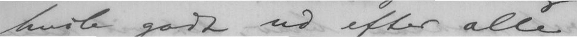hvile godt ud efter alle
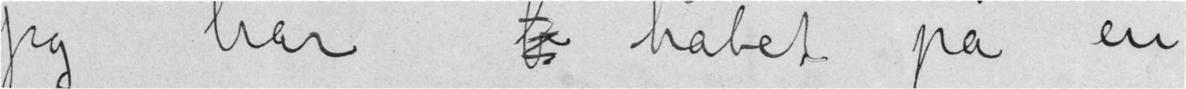jeg har håbet på en
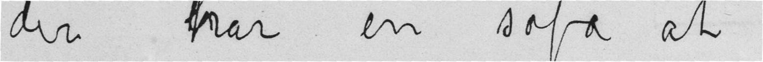der har en sofa at
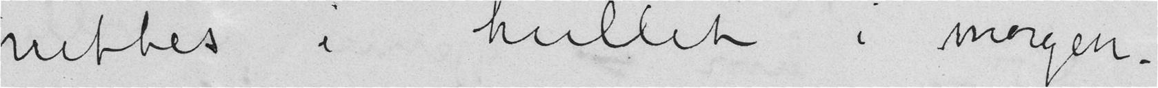puttes i hullet i morgen.
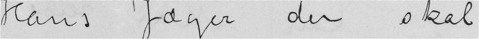Hans Jæger der skal
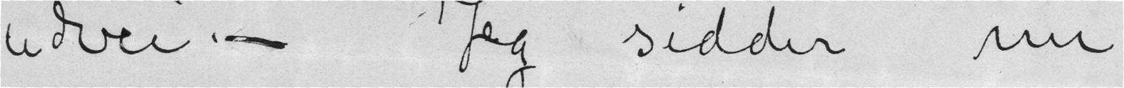udvei. – Jeg sidder nu
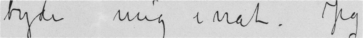byde mig inat. Jeg
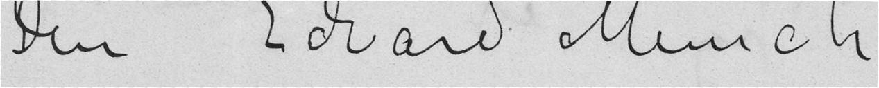Din Edvard Munch
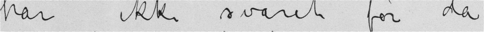har ikke svaret før da
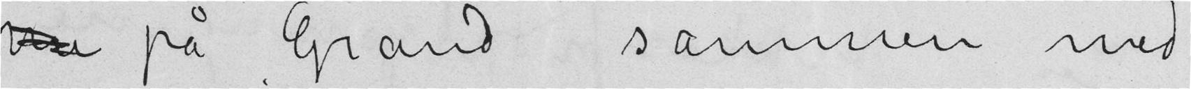på Grand sammen med
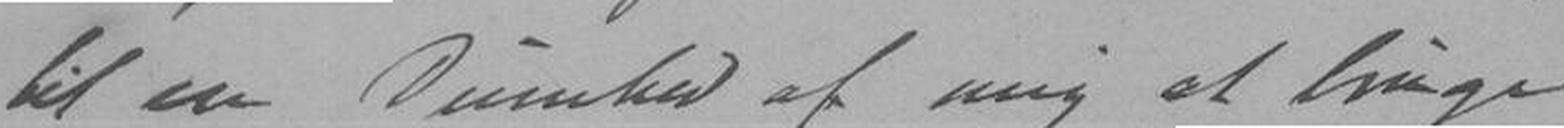til en Dumhed af mig at bruge
